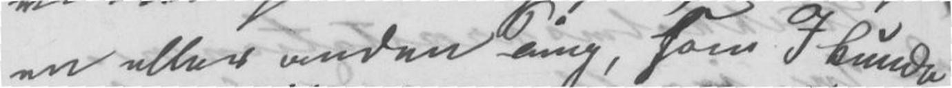en eller anden Ting; som I kunde
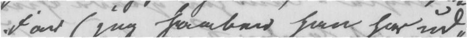Far (jeg haaber hun har ud-
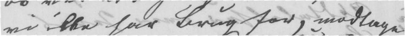vi ikke har brug for, modtage
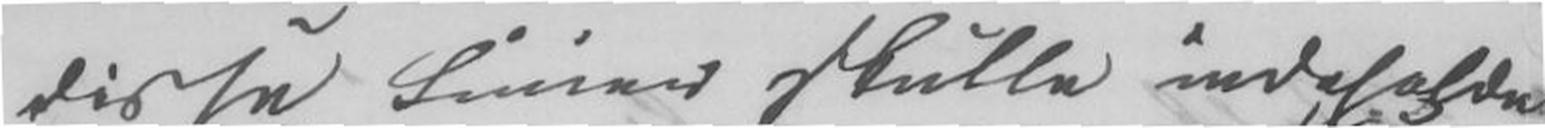disse Linier skulle indehalde
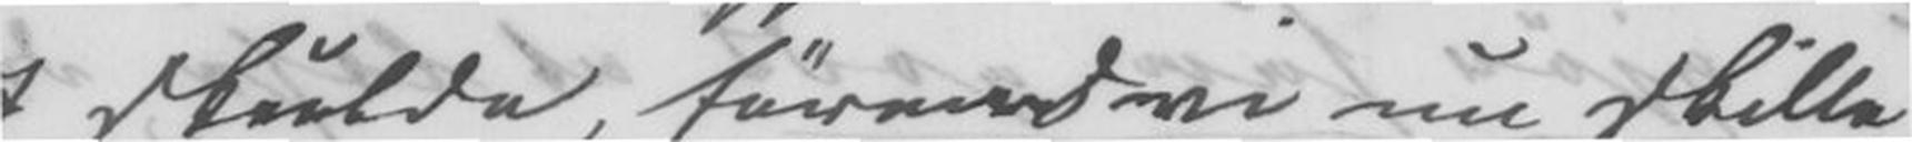I skulde, førend vi nu skille
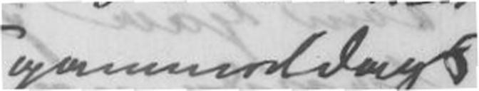gammeldagst
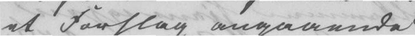et Førslag angaaende
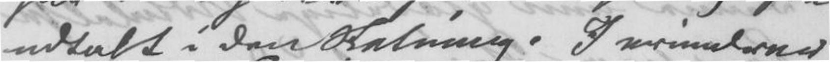udtalt i den Retning. I erindrer
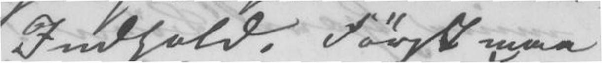Indfald. Først ma
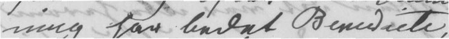ning har bedet Benedicte,
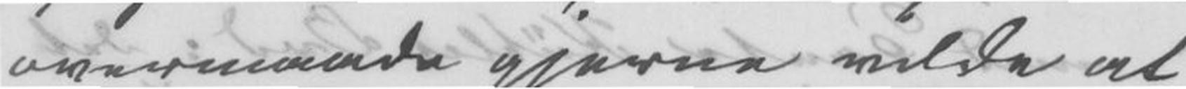overmaade gjerne nu vilde at
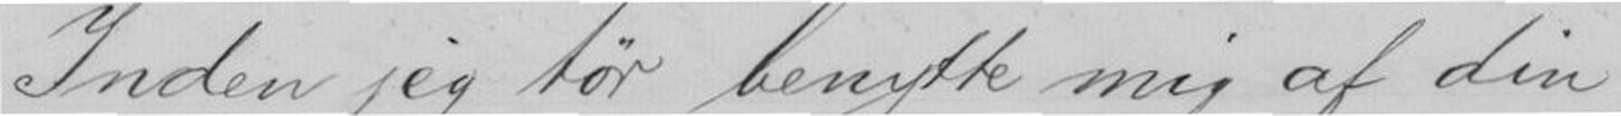Inden jeg tør benytte mig af din
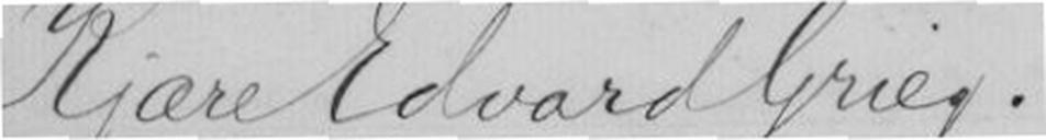Kjære Edvard Grieg !
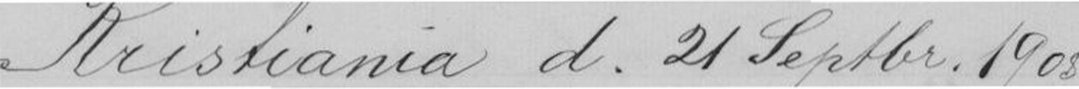Kristiania d. 21 Septbr. 1905.
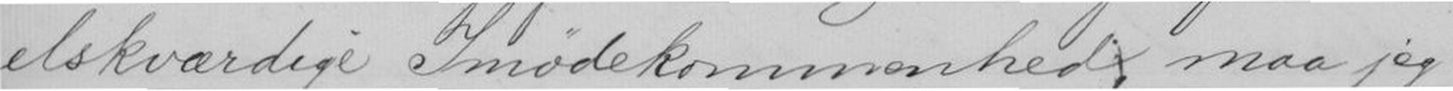elskværdige Imødekommenhed, maa jeg
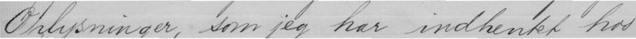Oplysninger, som jeg har indhentet hos
